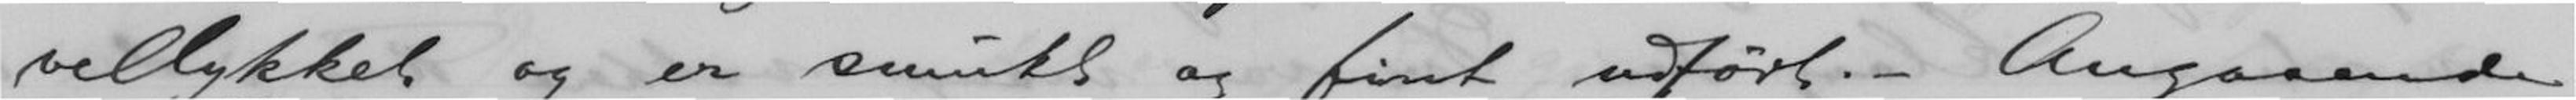vellykket og er smukt og fint Udført. - Angaaende
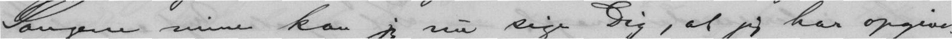Sangene mine kan jeg nu sige Dig, at jeg har opgivet
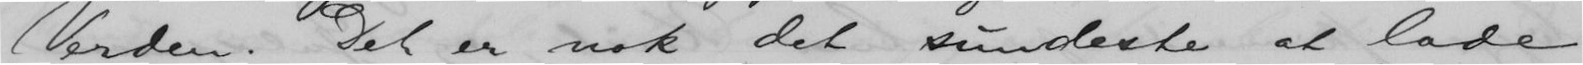Verden. Det er nok det sundeste at lade
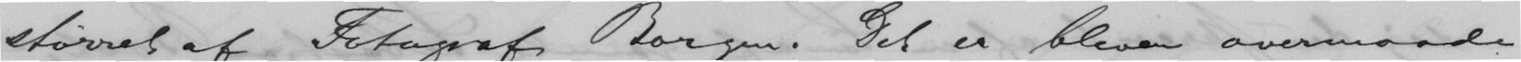størret af Fotograf Borgen. Det er bleven overmaade
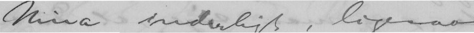Nina inderligt, ligesaa
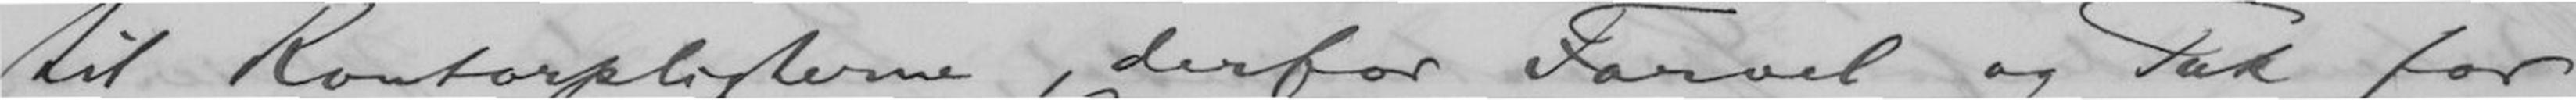til Kontorpligterne, derfor Farvel og Tak for
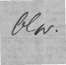Olav.
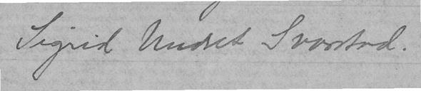Sigrid Undset Svarstad.
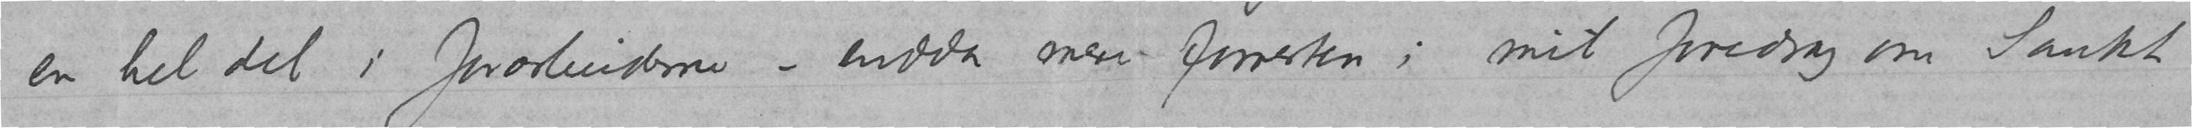en hel det i forarbeiderne – endda mere forresten i mit foredrag om Sankt
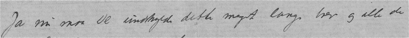Ja nu maa De undskylde dette meget lange brev og alle de
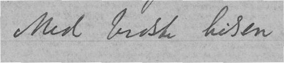Med bedste hilsen
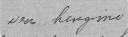Deres hengivne
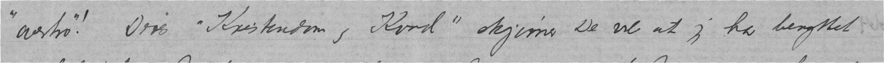"overtro"! Deres "Kristendom og Kvad" skjønner De vel at jeg har benyttet
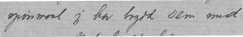spørsmaal jeg har brydd Dem med.
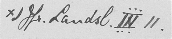x) Jfr. Landsl. III 11.
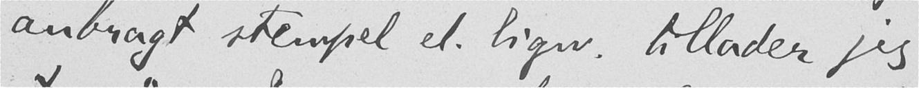anbragt stempel el. lign. tillader jeg
